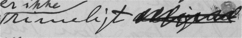rimeligt
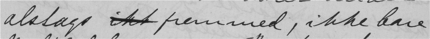alslags ikk fremmed, ikke bare
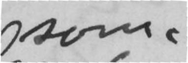som
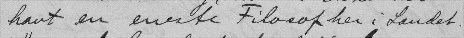havt en eneste Filosof her i Landet.
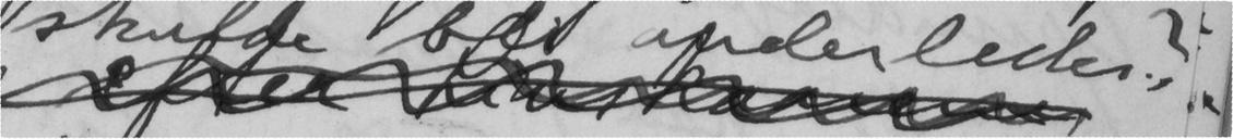skulde bli anderledes?
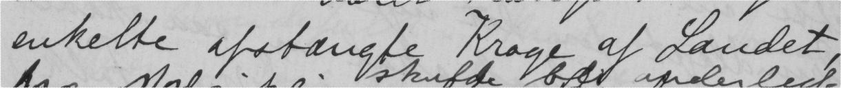enkelte afstængte Kroge af Landet,
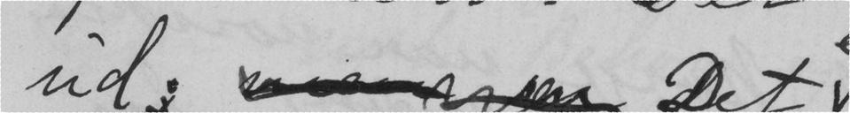ud.  Det
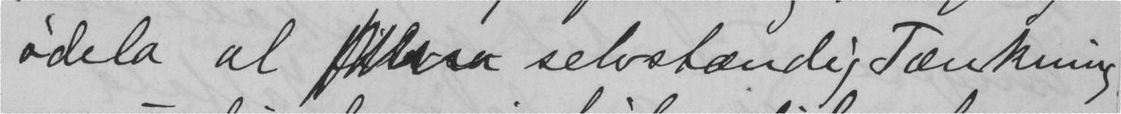ødela al  selvstændig Tænkning
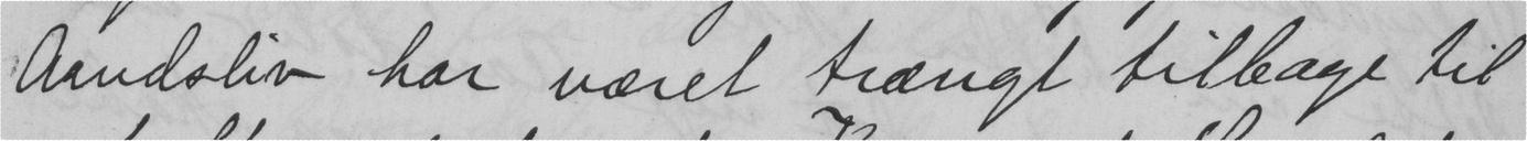Aandsliv har været trængt tilbage til
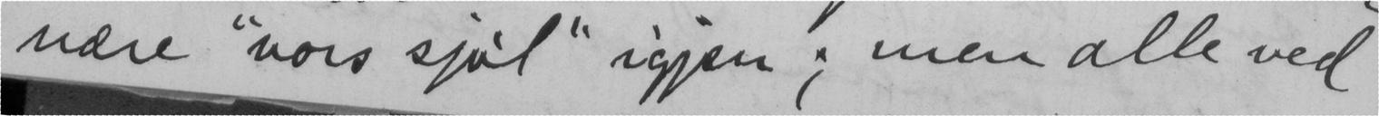være "vors sjøl" igjen; men alle ved
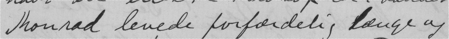Monrad levede forfærdelig længe og
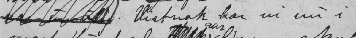Vistnok har vi nu i
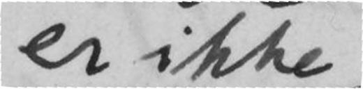er ikke
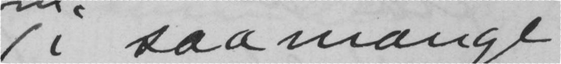i saa mange
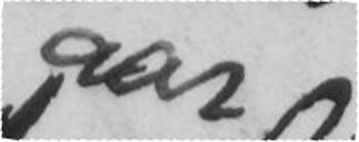aar
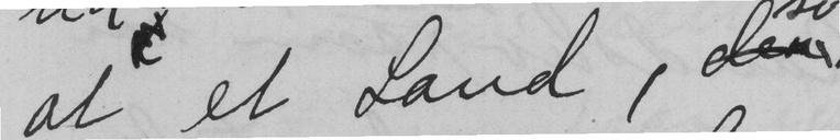at et Land, der
